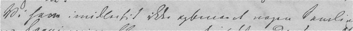Vi have imidlertid ikke opbevaret nogen Samling
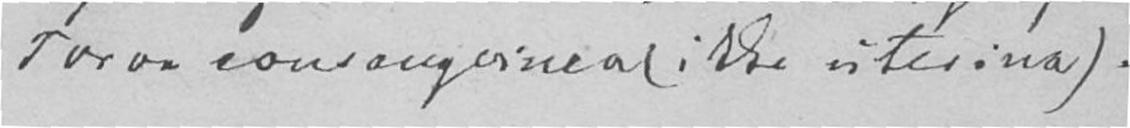Førre comangeina (ikke uterivat).
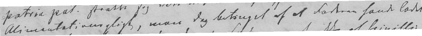Alimentationspligt, men dog betinget af at Faderen havde ladet
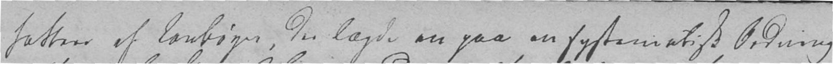fattere af Lovbøger, der lægde an paa en systematisk Ordning
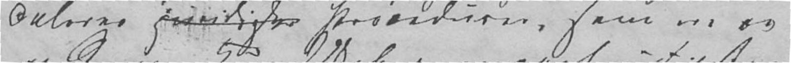Taleres juridiske Procedurer, som ere os
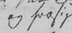og frasige
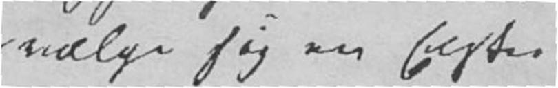vælge sig en Elsker
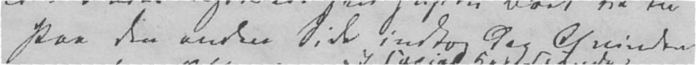Paa den anden Side indtog dog Qvinden
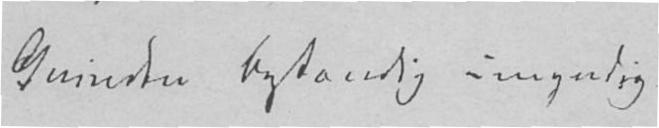Qvinden bestandig umyndig
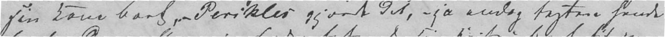sin Kone bort, Perikles gjorde det, ja endog testere hende
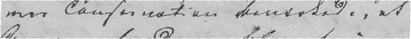mens Conservation bevirkede, at
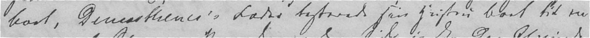bort, Demostehenes's Fader testerede sin Hustru bort til en
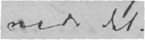nede det.
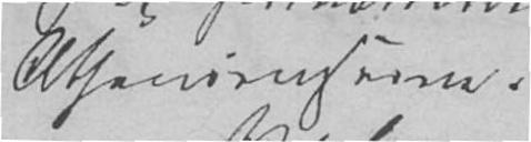Athenienserne.
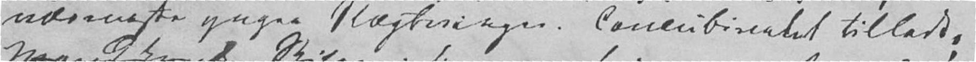nærmeste yngre Slægtninger. Concubinatet tillodes,
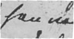han var
